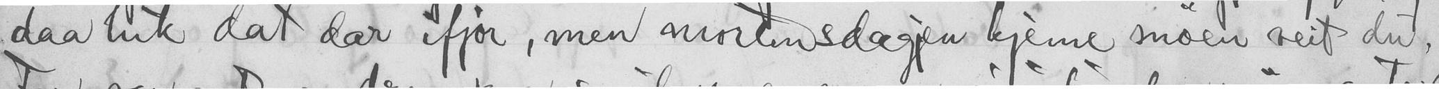daa hik dat dar igjor, men mortensdagjen kjeme snøen veit du,
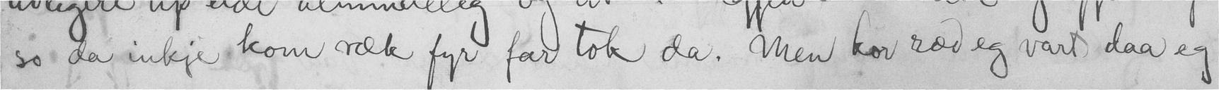so da inkje kom væk fyr far tok da. Men kor ræd eg vart daa eg
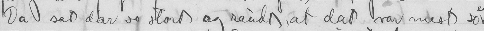Da sat dar so stort og raudt, at dat var mest so
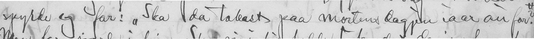spyrde eg Far: "Ska da takast paa mortens dagjen iaar au far?"
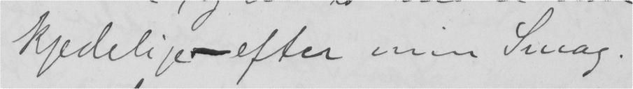kjedelige - efter min Smag.
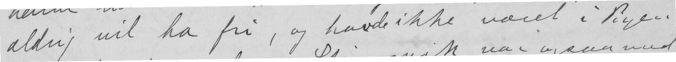aldrig vil ha fri, og havde ikke været i Byen
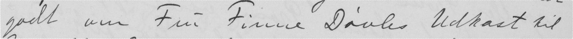godt om Fru Finne Døvles Udkast til
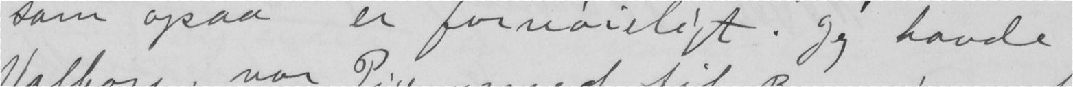som ogsaa er fornøieligt. Jeg havde
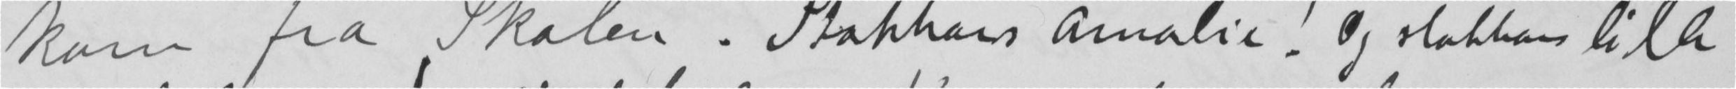kom fra Skolen. Stakhars Amalie! Og stakkars lille
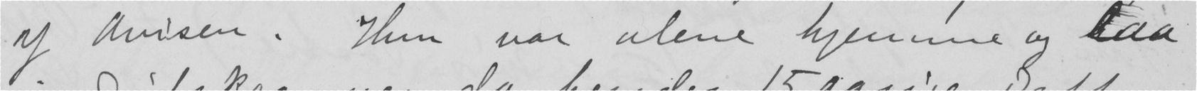af avisen. Hun var alene hjemme og laa
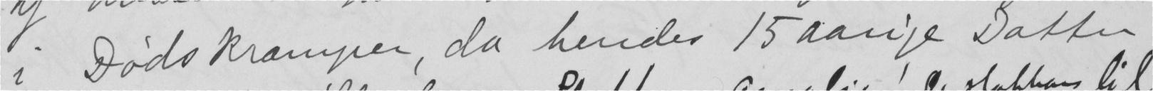i Dødskramper da hendes 15 Aarige Datter
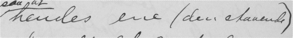hendes ene (den stavende)
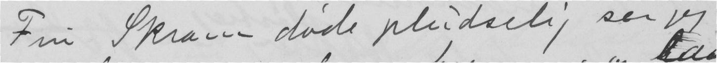Fru Skram døde pludselig ser jeg
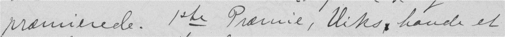præmierede. 1ste Præmie, Viks, havde et
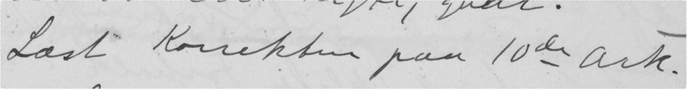Læst Korektur paa 10de Ark.
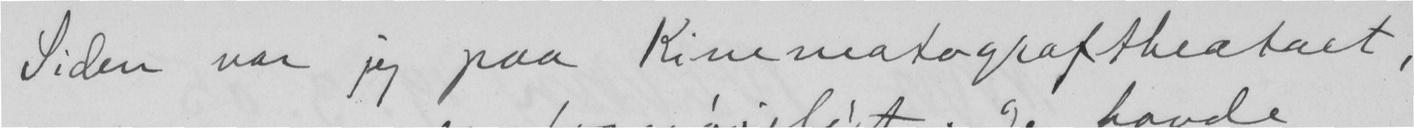Siden var jeg paa Kinematograftheatret,
